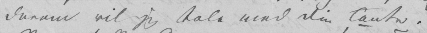derom vil jeg tale med Din Tante.
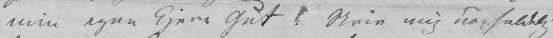min egen kjere Gut! Skriv mig uopholdelig
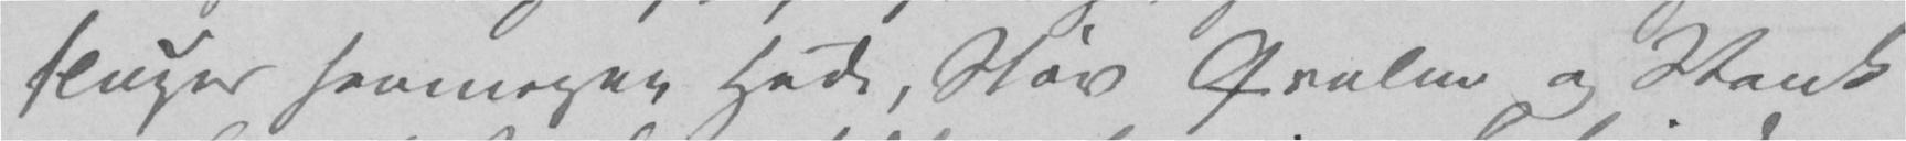sluger saamegen Hede, Støv Qvalm og Stank,
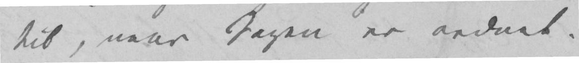til, naar Sagen er ordnet.
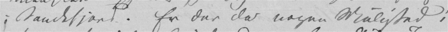i Sandefjord. Er der da nogen Mulighed i
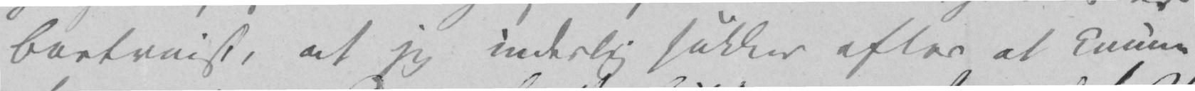bortreist, at jeg inderlig sukker efter at kunne
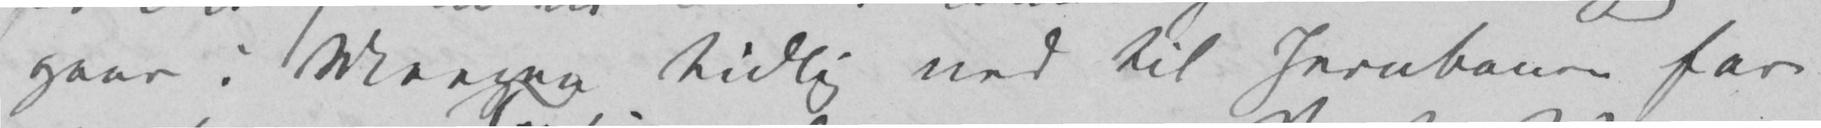gaar i Morgen tidlig ned til Jernbanen for
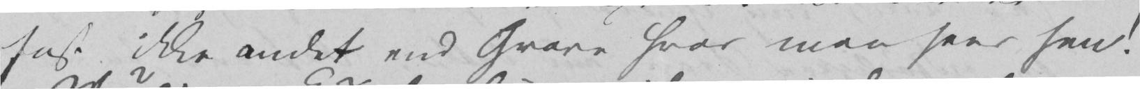fast ikke andet end Grave hvor man seer hen!
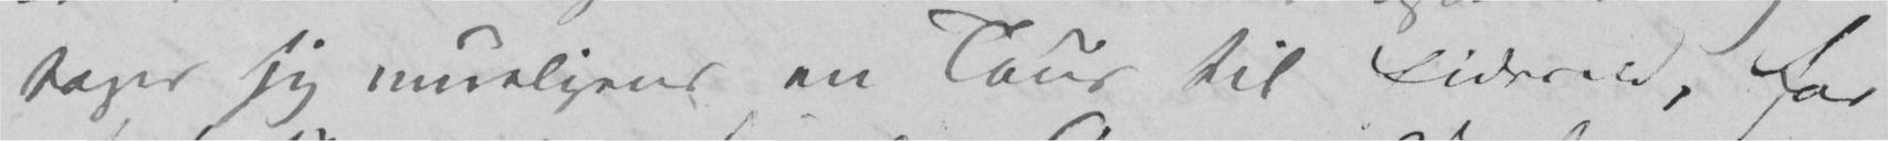tager jeg mueligens en Tour til Eidsvold, for
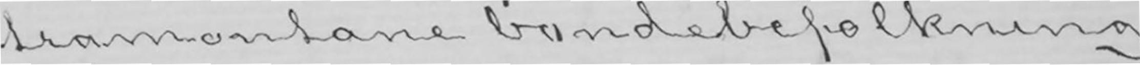tramontane bondebefolkning
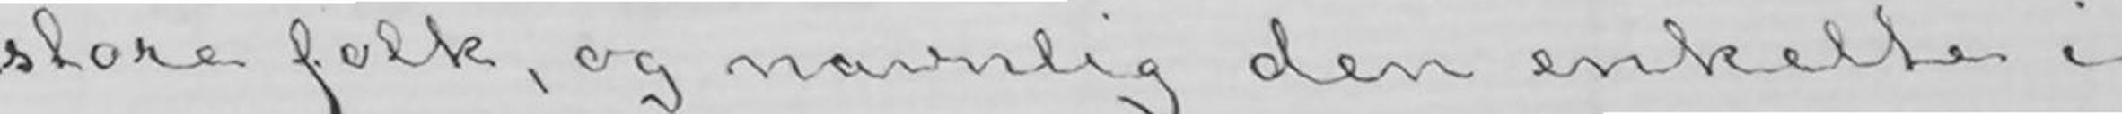store folk, og navnlig den enkelte i
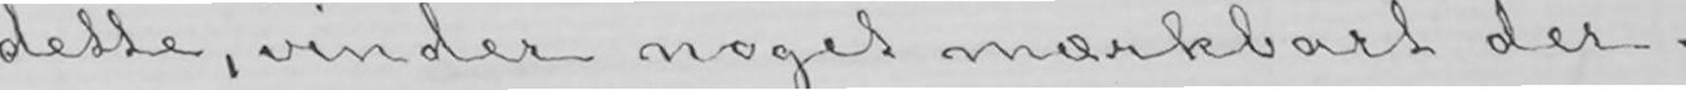dette, vinder noget mærkbart der-
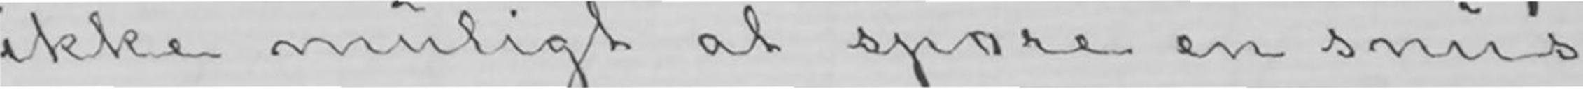ikke muligt at spore en snus
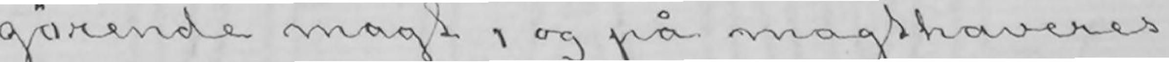gørende magt, og på magthaveres
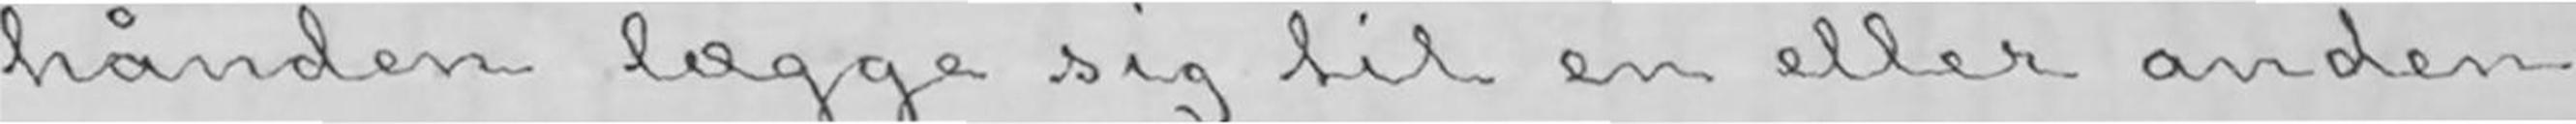hånden lægge sig til en eller anden
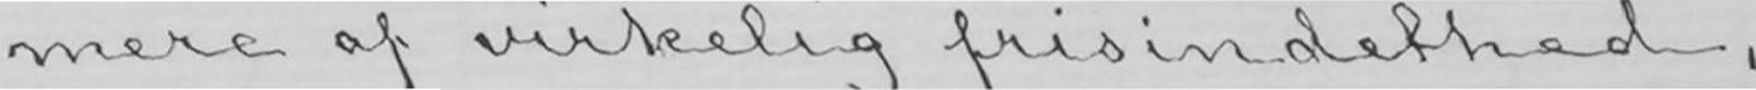mere af virkelig frisindethed,
